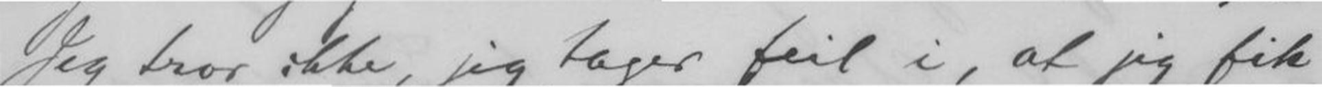Jeg tror ikke, jeg tager feil i, at jeg fik
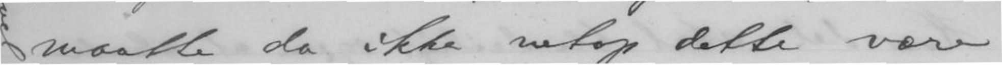maatte da ikke netop dette være
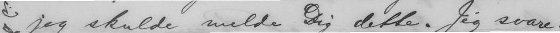jeg skulde melde Dig dette. Jeg svare-
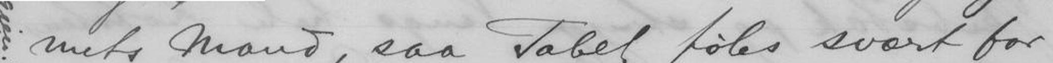mets Mand, saa Tabet føles svært for
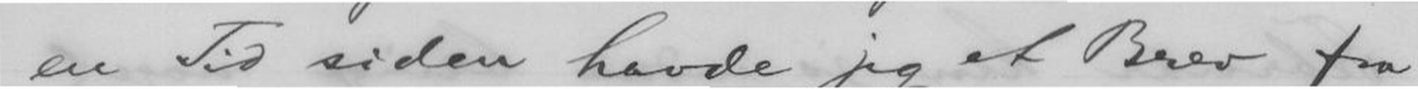en Tid siden havde jeg et Brev fra
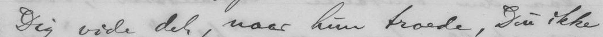Dig vide det, naar hun troede, Du ikke
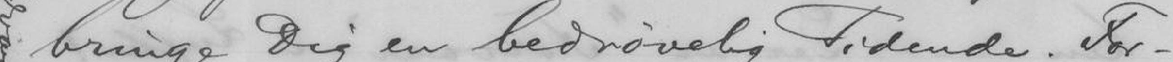bringe Dig en Bedrøvelig Tidende. For
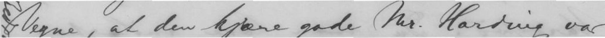Vegne, at den kjære gode Mr. Harding var
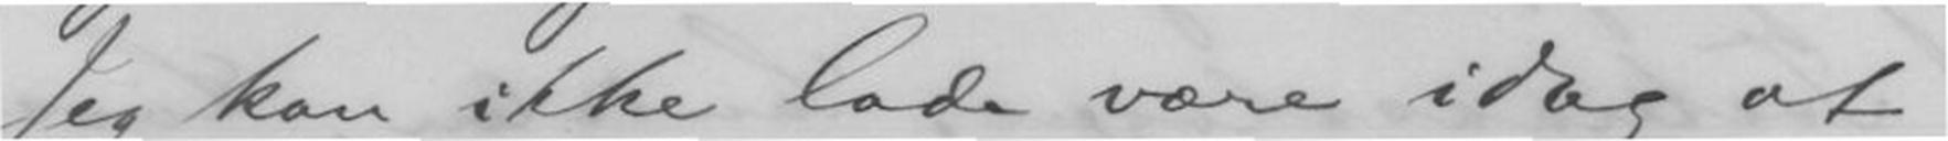Jeg kan ikke lade være idag at
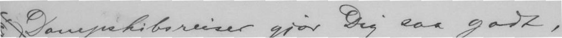Dampskibsreiser gjør Dig saa godt,
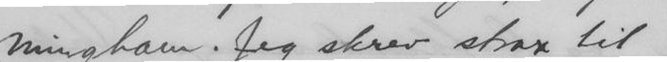mingham. Jeg skrev strax til
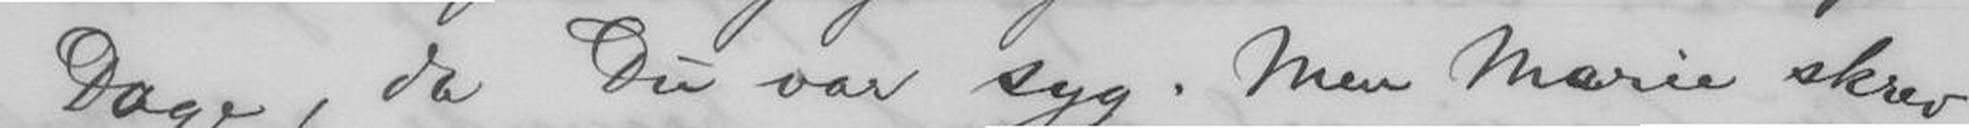Dage, da Du var syg. Men Marie skrev
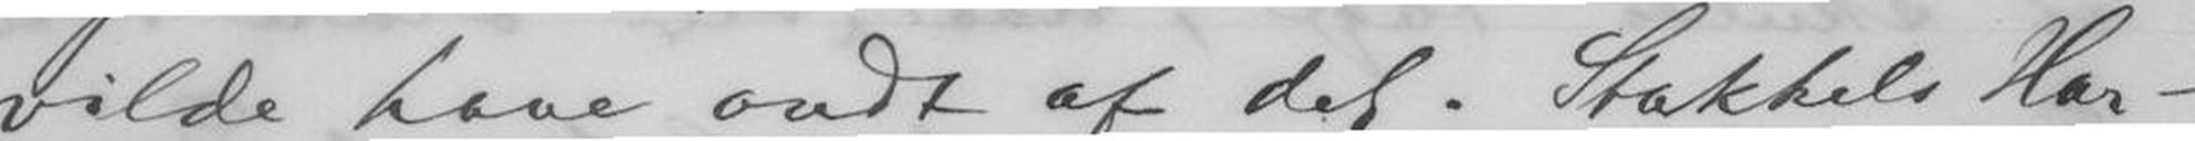vilde have ondt af det. Stakkels Har-
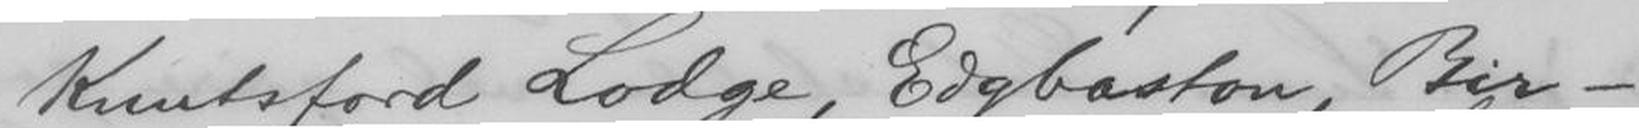Knutsford Lodge, Edgbaston, Bir-
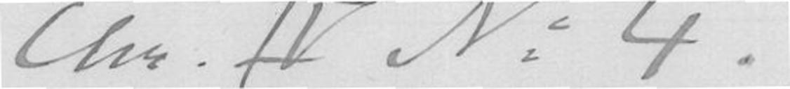Chr. IV N: 4.
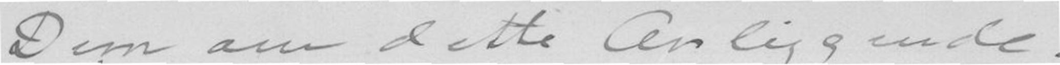Dem om dette Anliggende.
